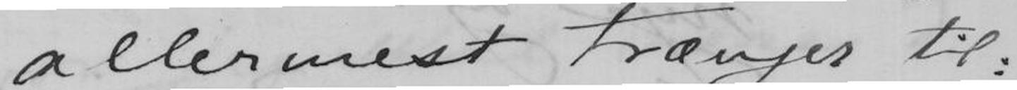aller mest trænger til:
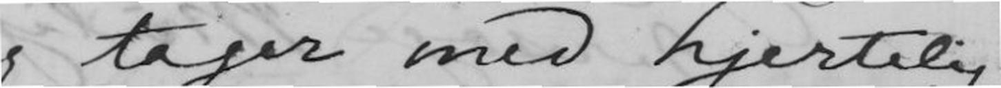jeg tager med hjertelig
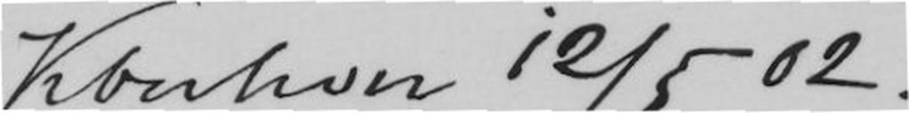Kbnhvn 12/5 02.
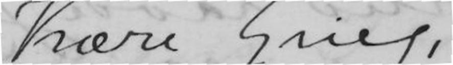Kjære Grieg.
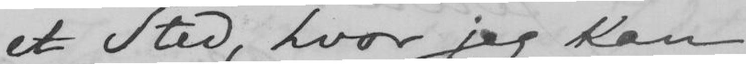et Sted, hvor jeg kan
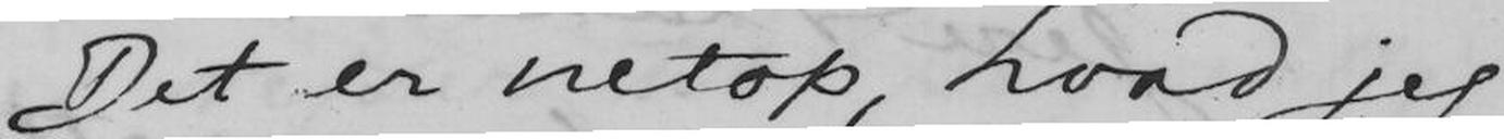Det er netop, hvad jeg
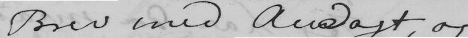Brev med Andagt, og
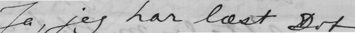Ja, jeg har læst Dit
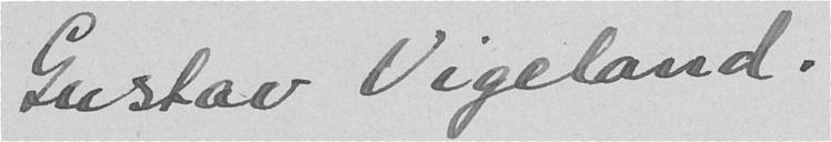Gustav Vigeland
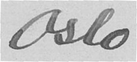Oslo
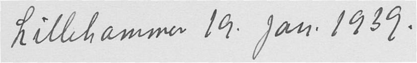Lillehammer 19. jan. 1939.
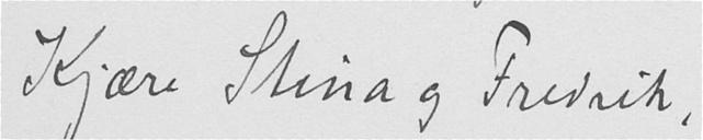Kjære Stina og Fredrik,
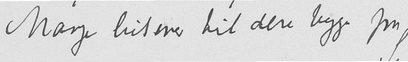Mange hilsener til dere begge fra
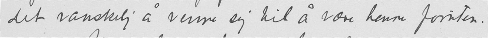det vanskelig å venne sig til å være henne foruten.
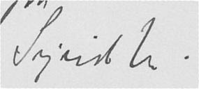Sigrid U.
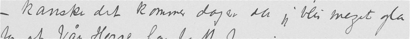- kanske det kommer dager da jeg blir meget gla
